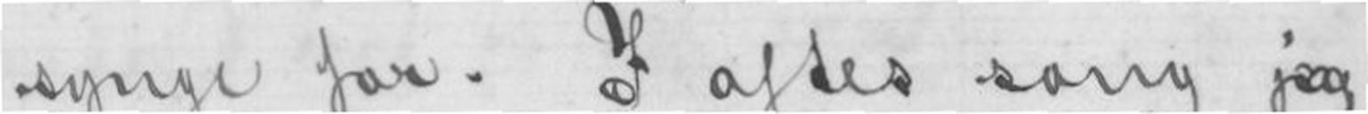synge for. I aftes sang jeg
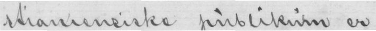stiniansiske publikum er
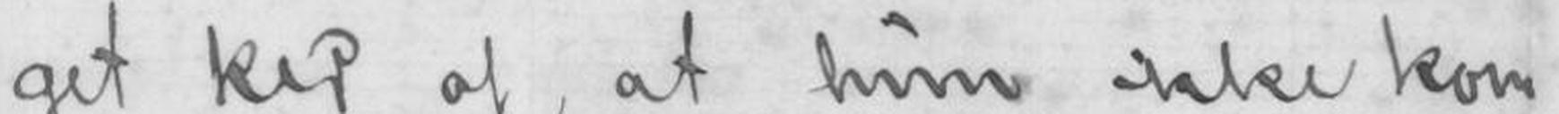get ked af, at hun ikke kom
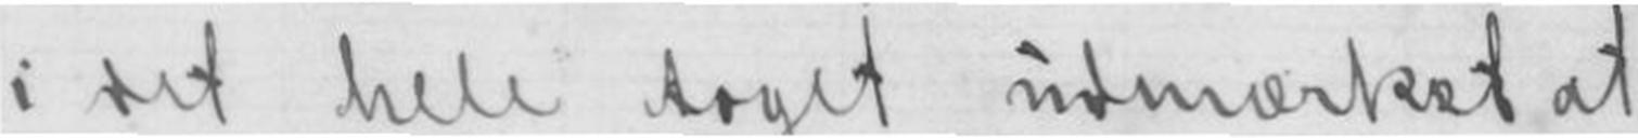i det hele taget udmærket at
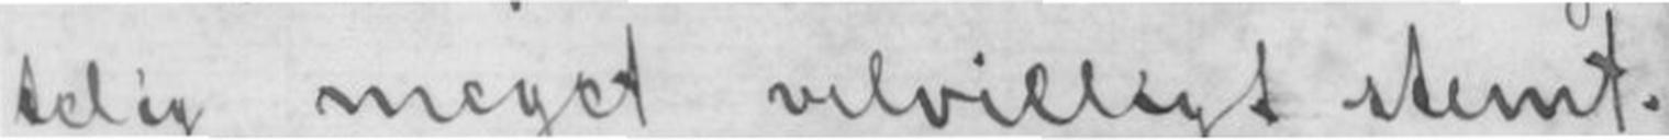telig meget velvilligt stemt.
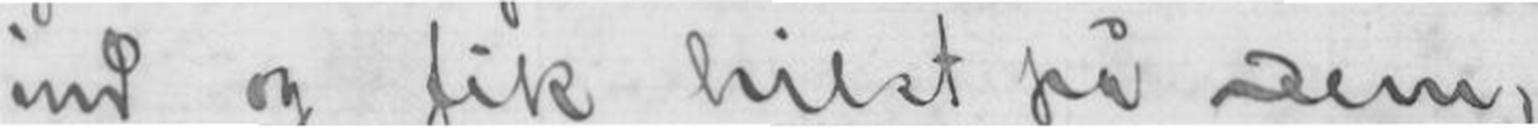ind og fik hilst på Dem,
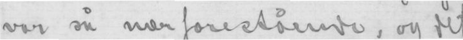var så nær forestående, og det
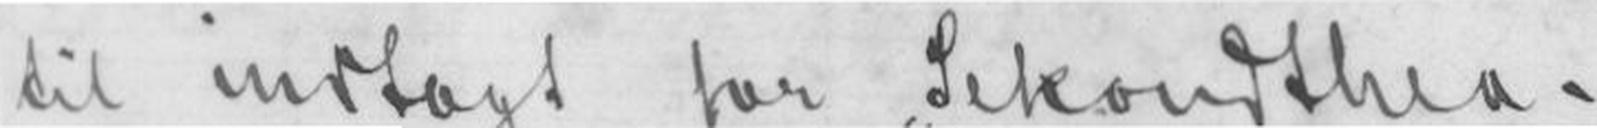til indtægt for Sekondthea-
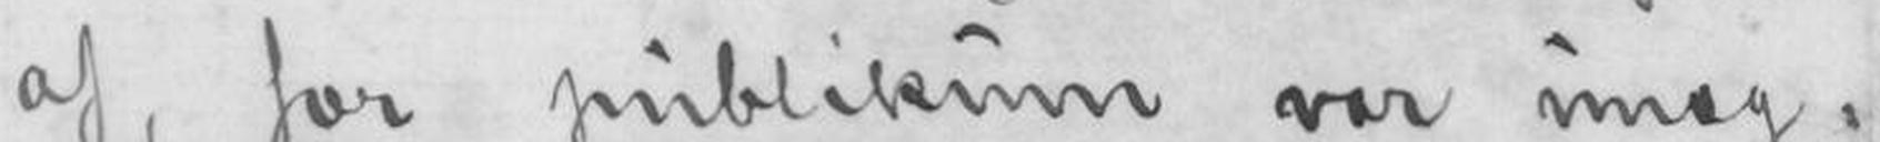af, for publikum var unæg-
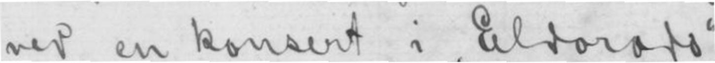ved en konsert i Eldorado
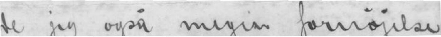de jeg også megen fornøjelse
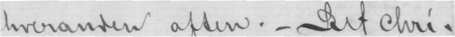hveranden aften.- Det Chri-
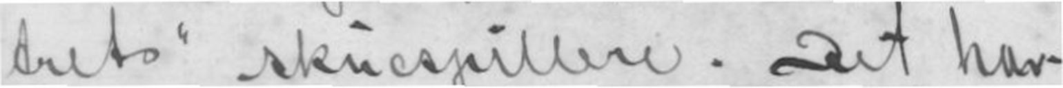trets skuespillere. Det hav-
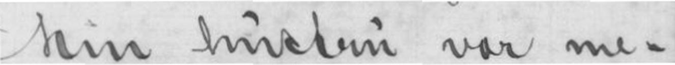Min hustru var me-
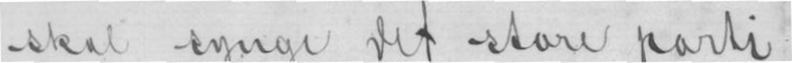skal synge det store parti
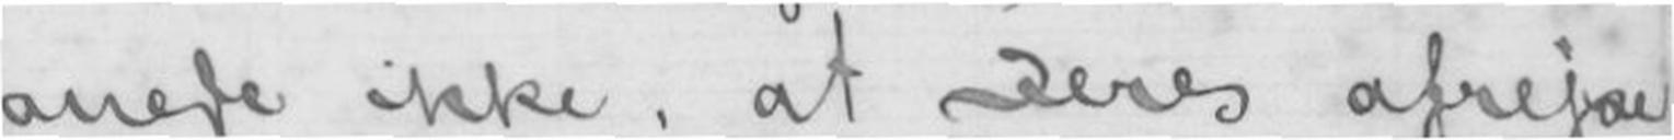anede ikke, at Deres afrejse
